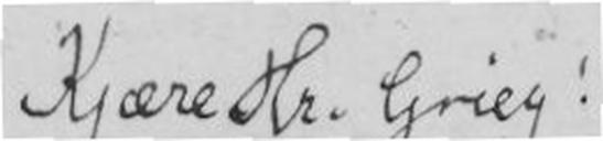Kjære Grieg!
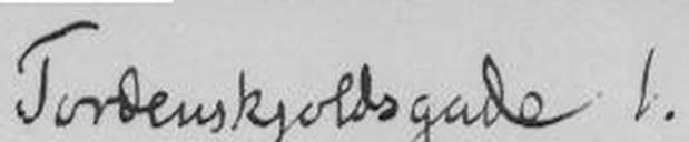Tordenskjoldsgade 1.
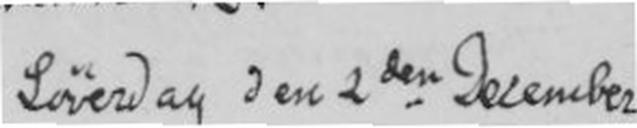Løverdag den 2den December
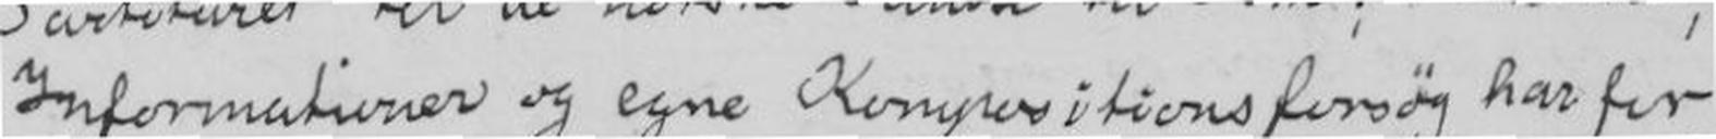Informationer og egne Kompositionsforsøg har for-
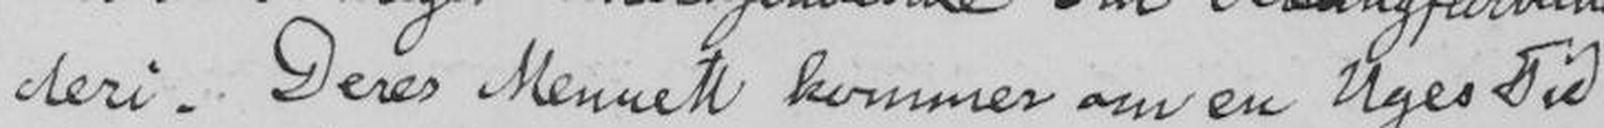deri. Deres Menuett kommer om en Uges Tid
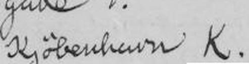Kjøbenhavn K.
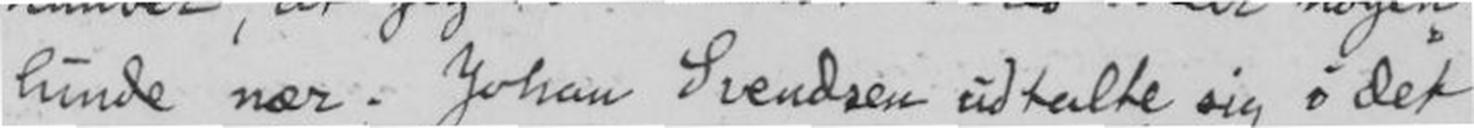lunde nær; Johan Svendsen udtalte sig i det
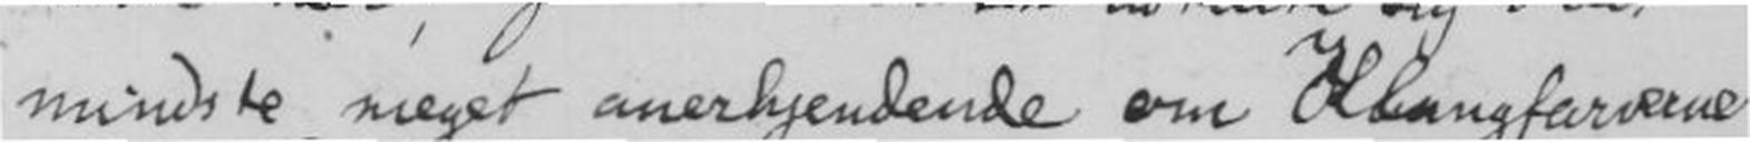mindste meget anerkjendende om Klangfarverne
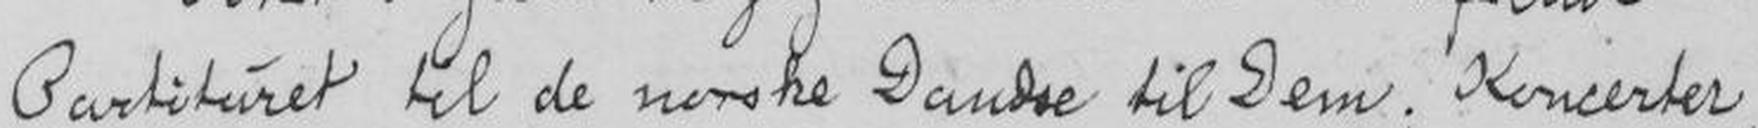Partituret til de norske Dandse til Dem, Koncerter,
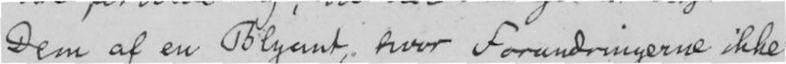Dem af en Blyant, Hvor Forundringerne ikke
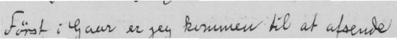Først i Gaar er jeg kommet til at afsende
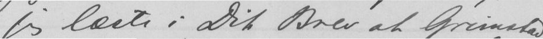jeg læste i Dit Brev at Grimstad
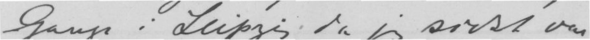Gange i Leipzig da jeg sidst var
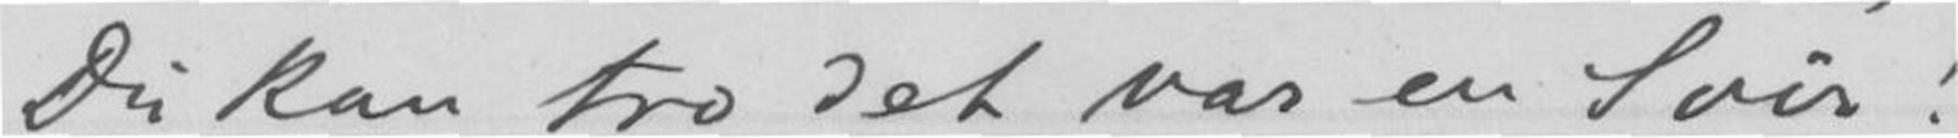Du kan tro det var en Svir!
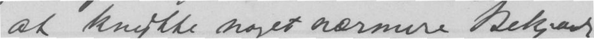at knytte noget nærmere Bekjent-
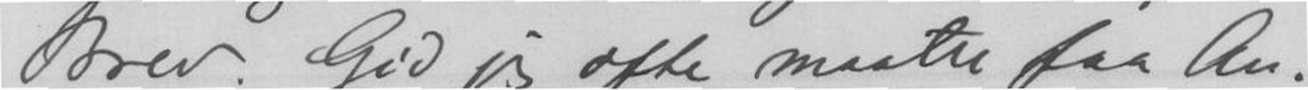Brev. Gid jeg ofte maatte faa An-
